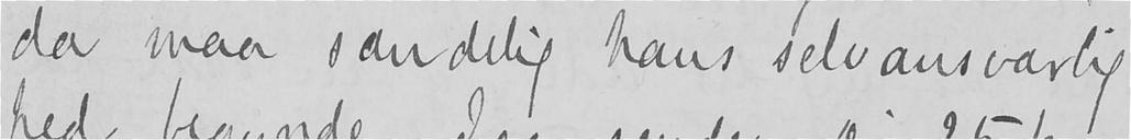da maa sandelig hans selvansvarlig-
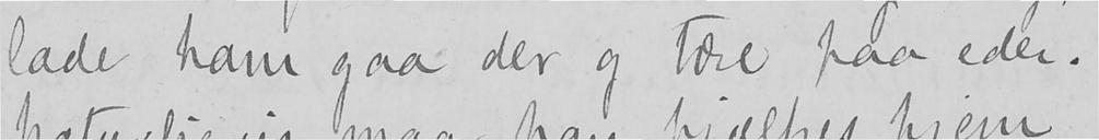lade ham gaa der og tære paa eder.
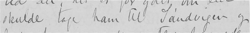skulde tage ham til Sandvigen og
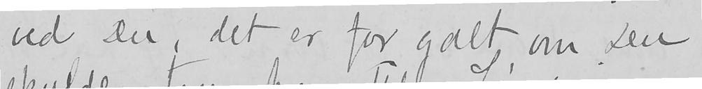ved Du, det er for galt om Du
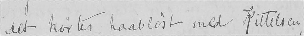Det hørtes haabløst med Kittelsen,
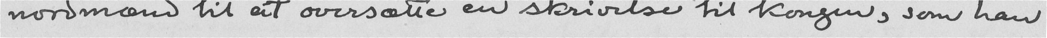nordmænd til at oversætte en skrivelse til kongen, som han
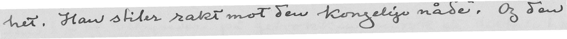het. Han stiler rakt mot den "kongelige nåde". Og den
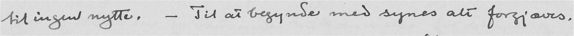til ingen nytte. - Til at begynde med synes alt forgjæves.
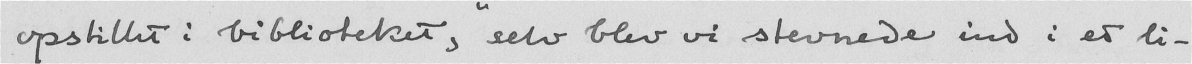opstillet i biblioteket, "selv blev vi stevnede ind i et li-
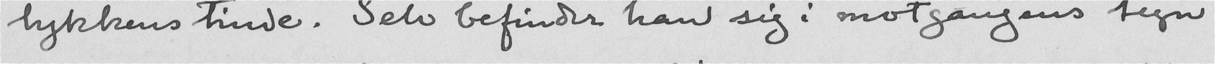lykkens tinde. Selv befinder han sig i motgangens tegn
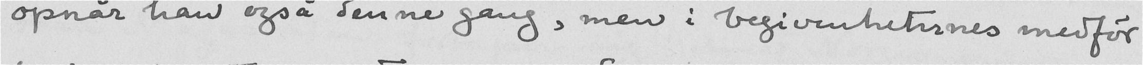opnår han også denne gang, men i begivenheternes medfør
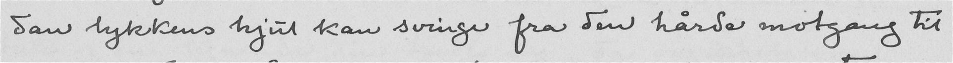dan lykkens hjul kan svinge fra den hårde motgang til
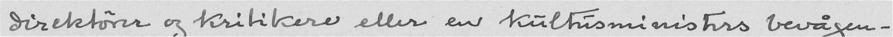direktører og kritikere eller en kulturministers bevågen-
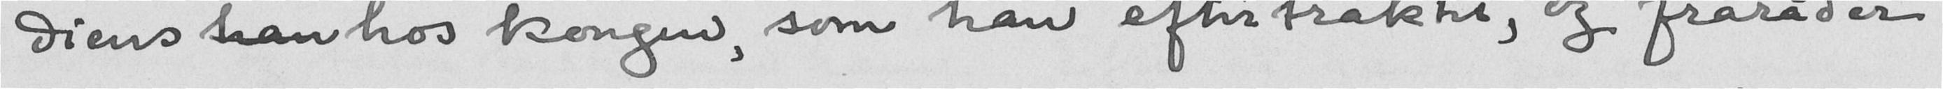diens han hos kongen, som han eftertraktet, og fraråder
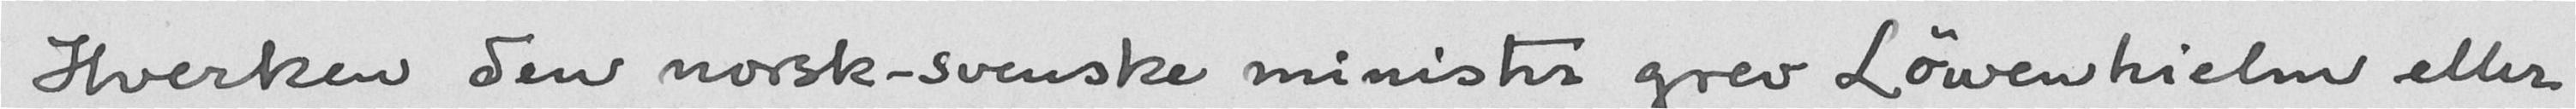Hverken den norsk-svenske minister grev Løwenhielm eller
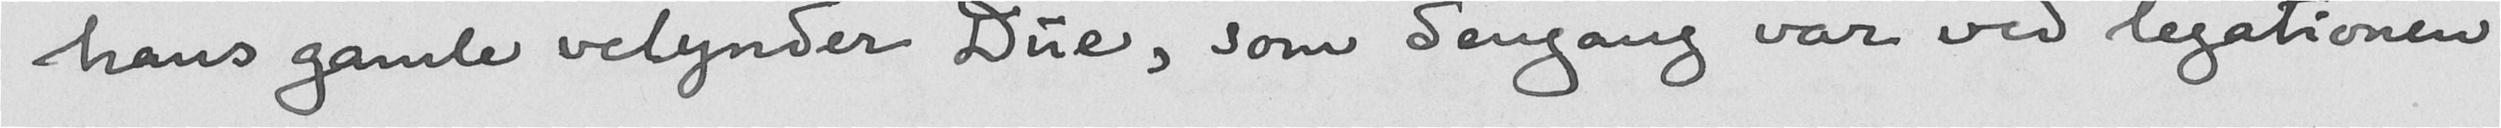hans gamle velynder Due, som dengang var ved legationen
